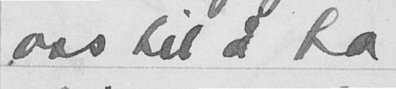oss til å ta
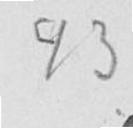93
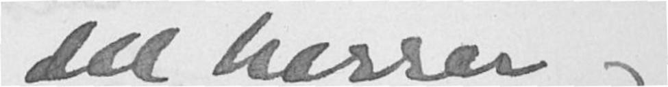del herrer og
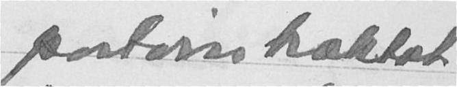partiene takket
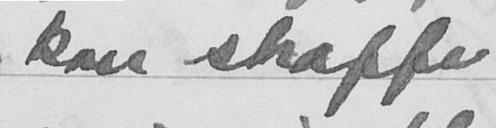kan straffe
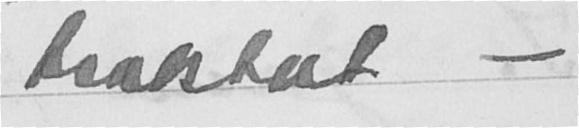traktat -
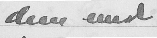dem med
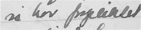vi har forpliktet
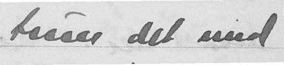truer det med
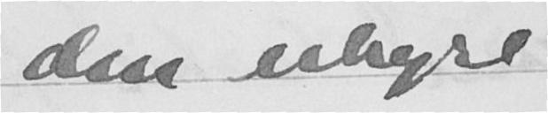den uhyre
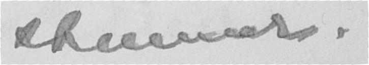skimnar.
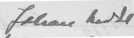Johan hadde
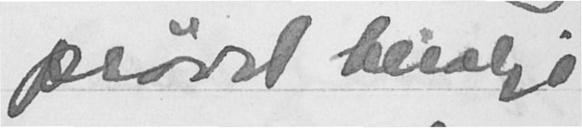prøvd besegle
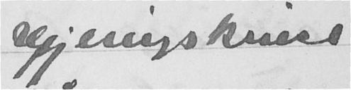regjeringskuiel
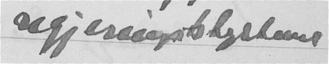regjeringskbystemns
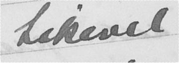Likevel
